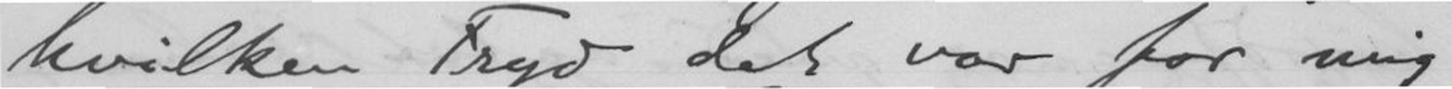hvilken Fryd det var for mig
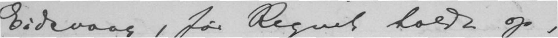Eidsvaag, før Regnet holdt op,
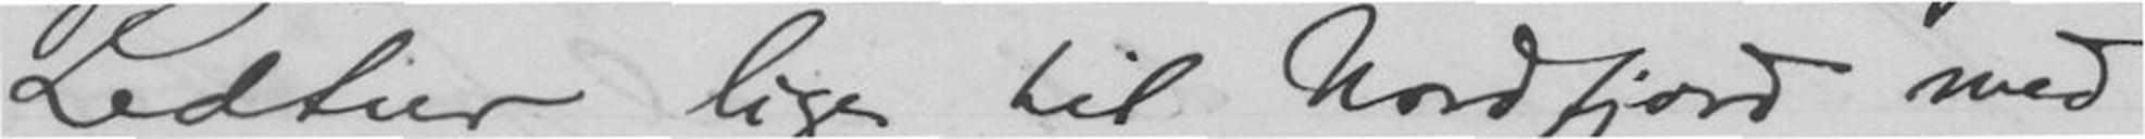Ledtur lige til Nordfjord med
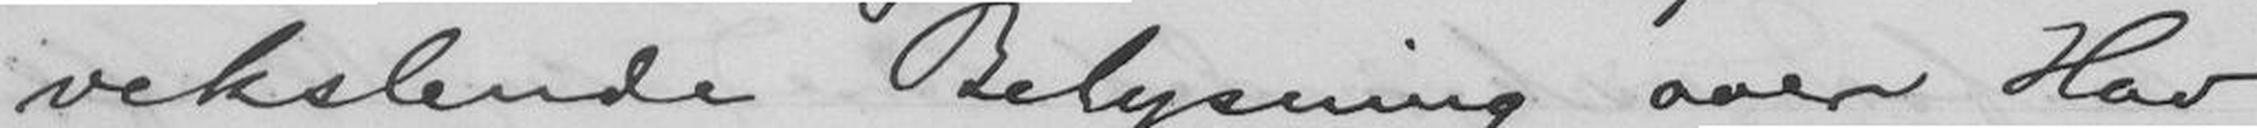vekslende Belysning over Hav
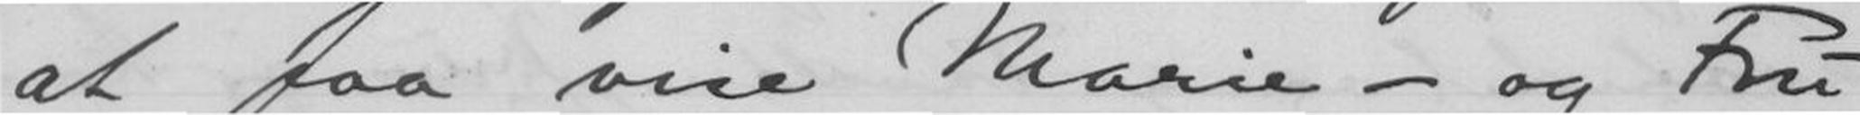at faa vise Marie - og Fru
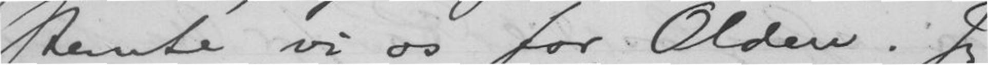stemte vi os for Olden. Jeg
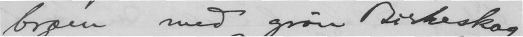bræen med grøn Birkeskog
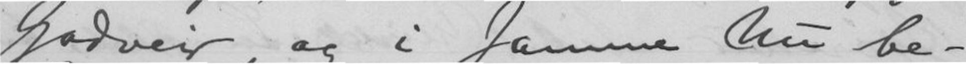Godveir, og i samme Nu be-
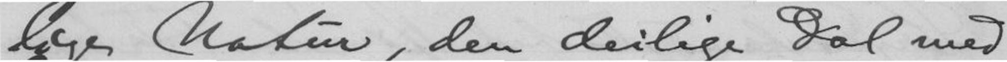lige Natur, den deilige Dal med
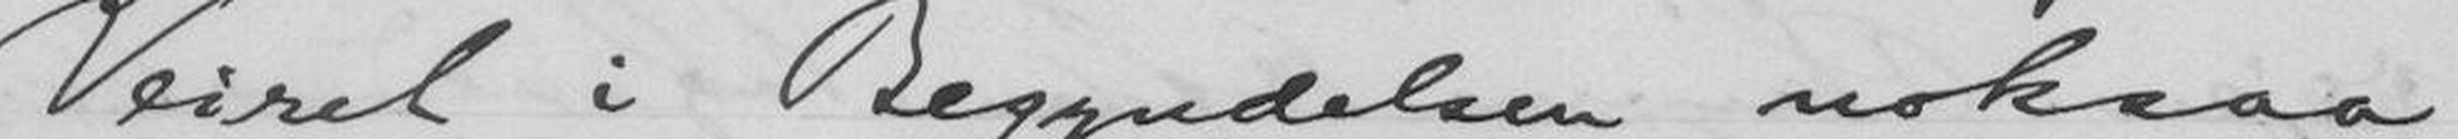Veiret i Begyndelsen noksaa
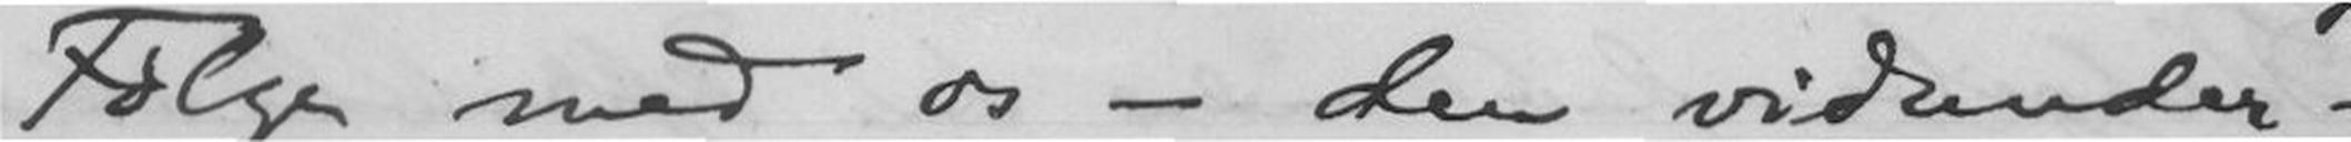Følge med os - den vidunder
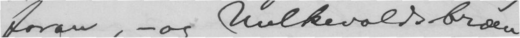foran, - og Melkevoldsbræen
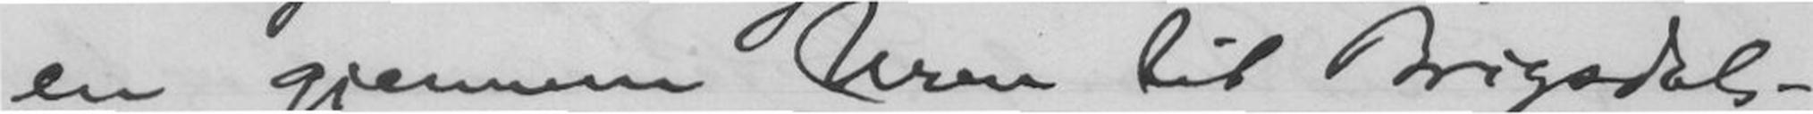en gjennem Urene til Brigsdals-
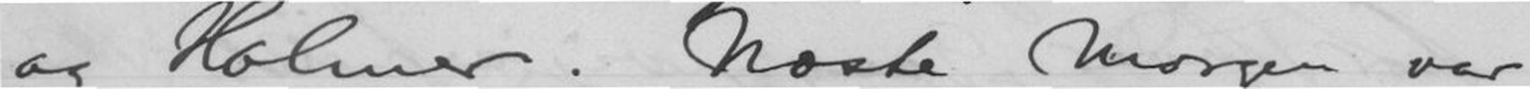og Holmer. Næste Morgen var
